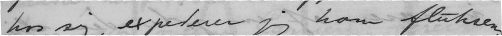hos sig, expederer jeg ham fluksens
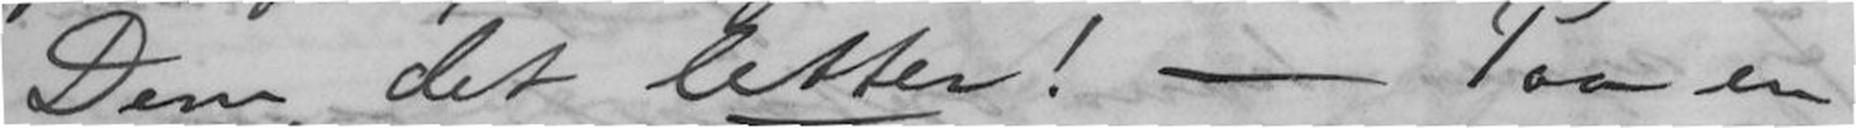Dem, det letter! - Paa en
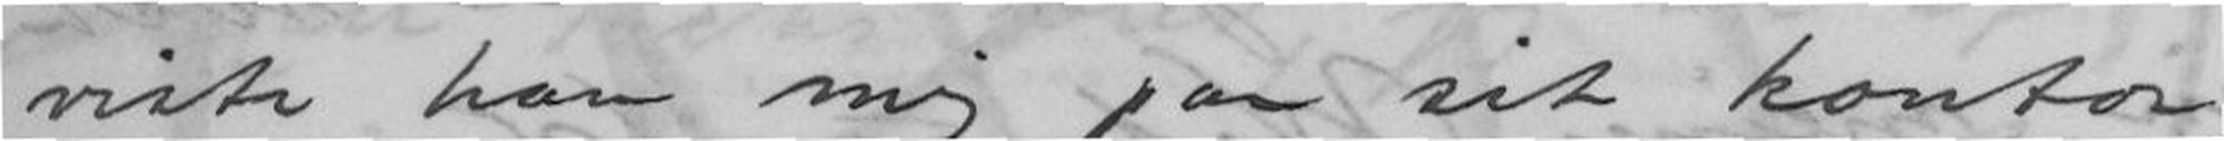viste han mig paa sit kontor,
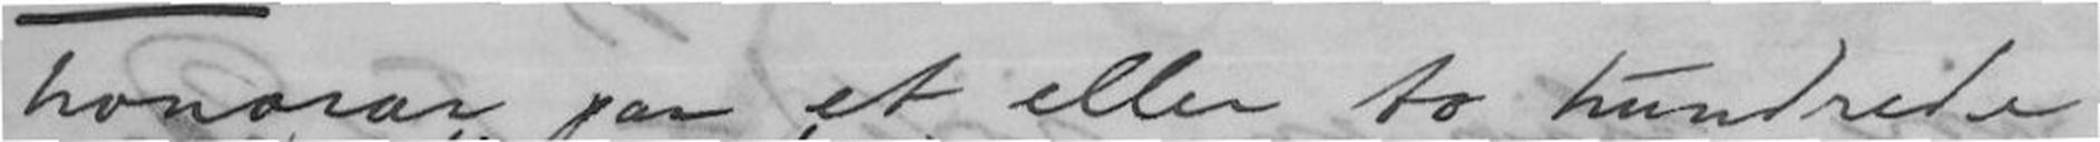honorar paa et eller to hundrede
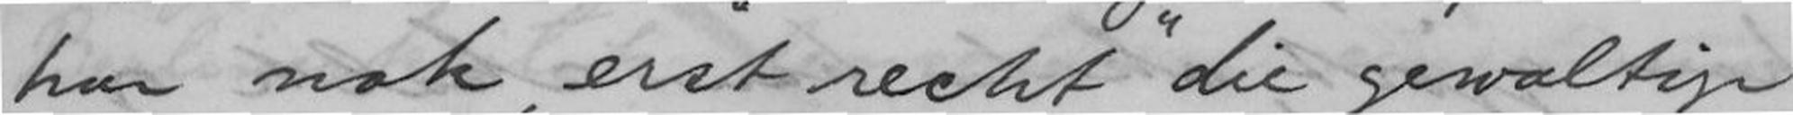har nok "erst recht" die gewaltige
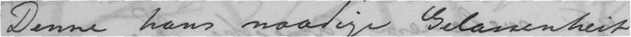Denne hans naadige Gelassenheit
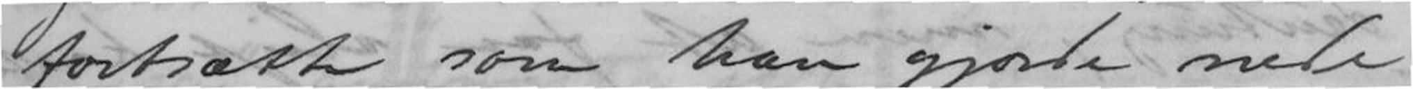fortsætte som han gjorde nede
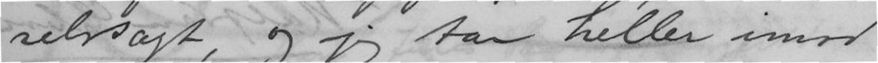selvsagt, og jeg tar heller imod
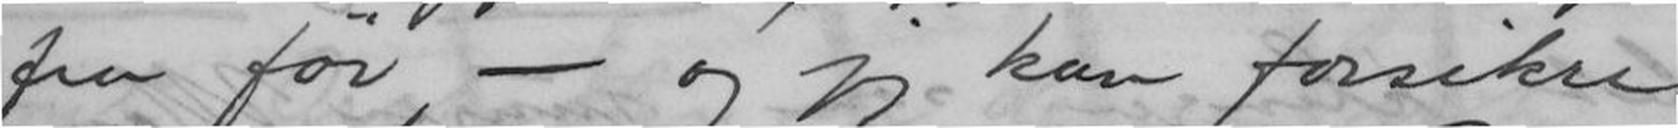fra för, - og jeg kan forsikre
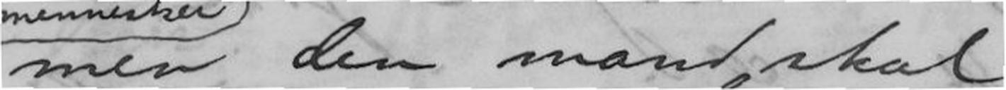men den mand skal
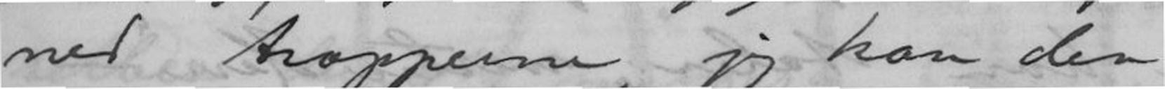ned trapperne, jeg kan den
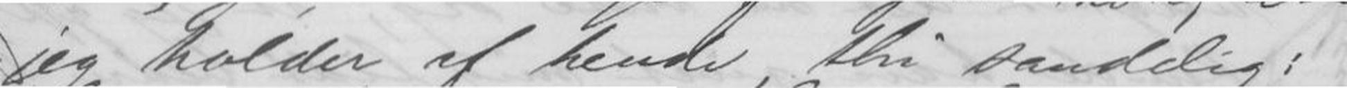jeg holder af hende, thi sandelig,
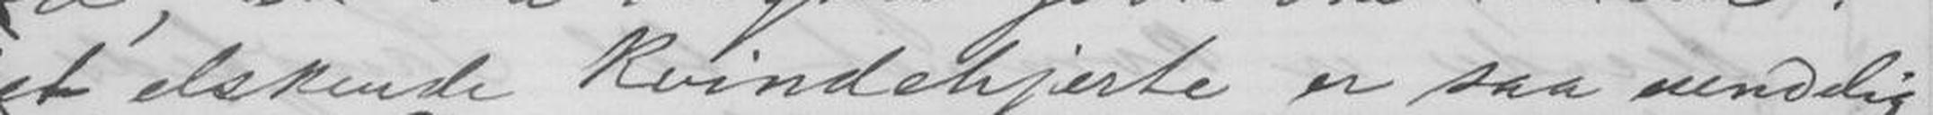et elskende Kvindehjerte er saa uendelig
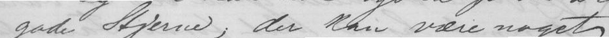gode Stjerne, der kan være noget
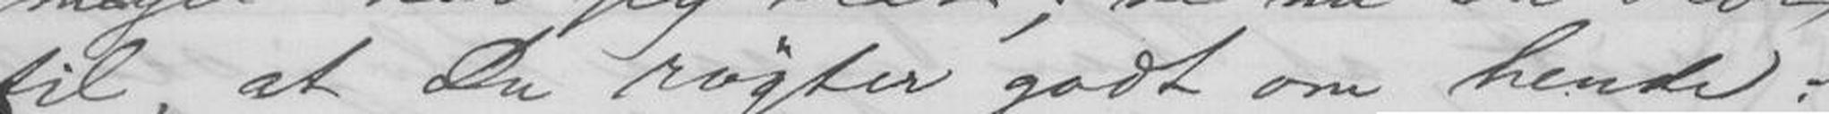til, at Du røgter godt om hende:
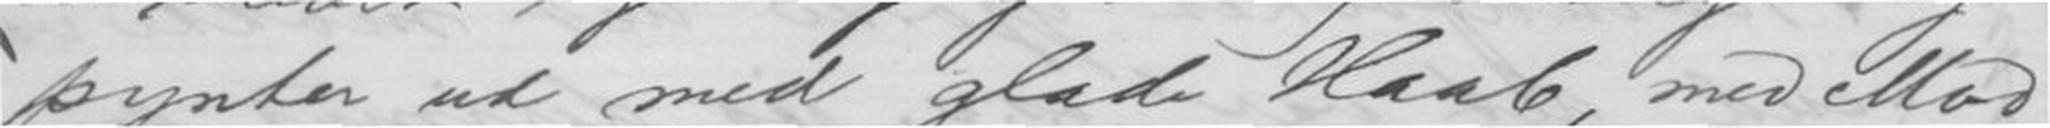pynter ud med glade Haab, med Mod
